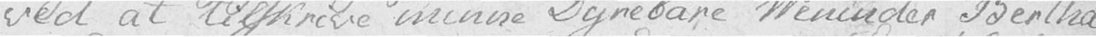ved at tilskrive minne Dyrebare Venider Bertha
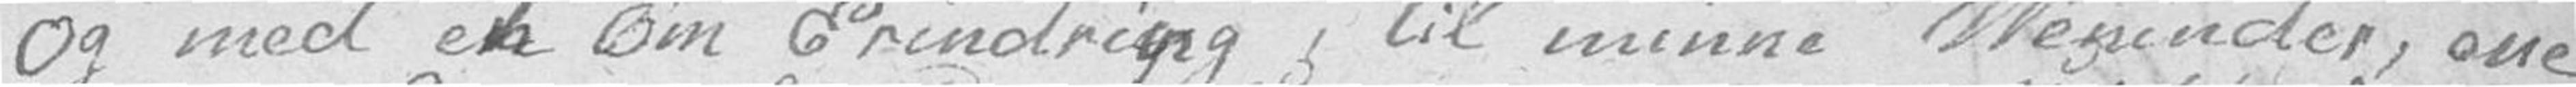Og med en Øm Erindring, til minne Veninder, ene
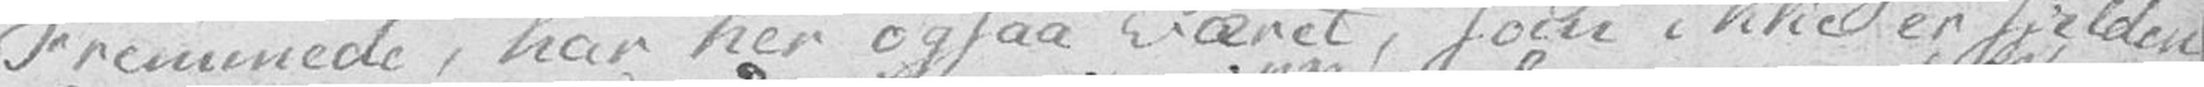Fremmede, har her ogsaa været, som ikke er sjelden
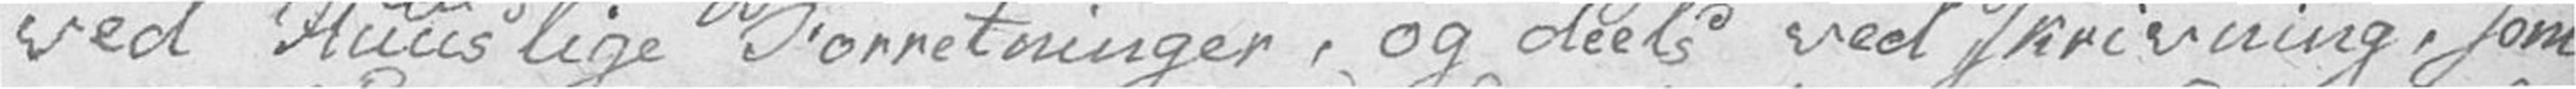ved Huuslige Forretninger, og deels ved skrivning, som
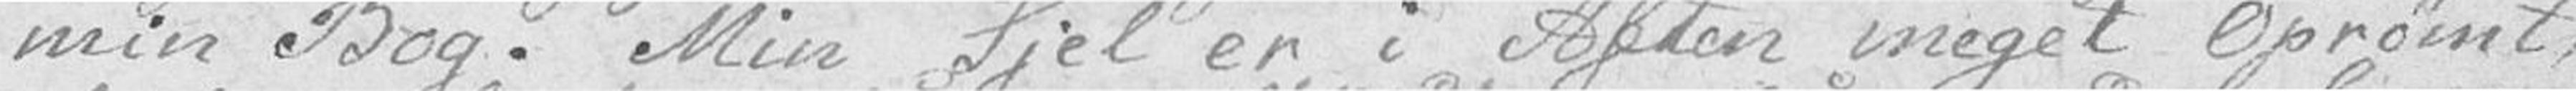min Bog. Min Sjel er i Aften meget Oprømt,
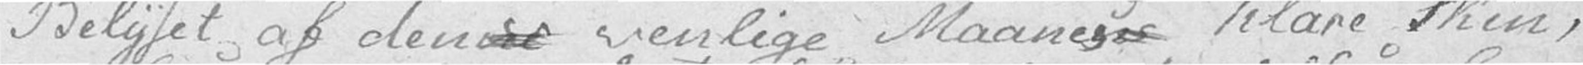Belyset af denne venlige Maanens  Klare Skin,
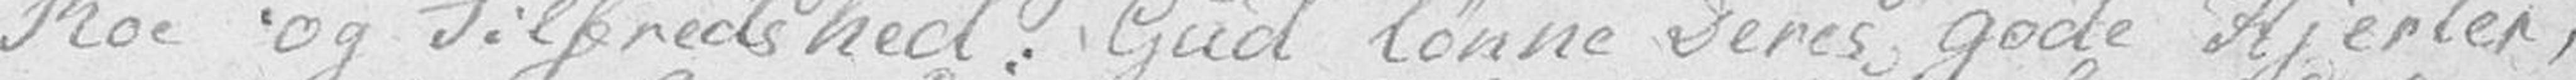Roe og Tilfredshed. Gud lønne Deres gode Hjerter,
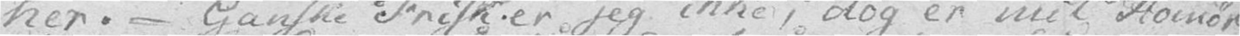her. - Ganske Frisk er jeg ikke, dog er mit Homør
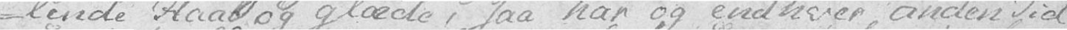-lende Haab og glæde, saa har og endhver anden Tid
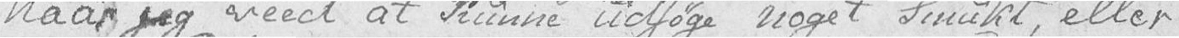naar jeg veed at kunne udsøge noget Smukt, eller
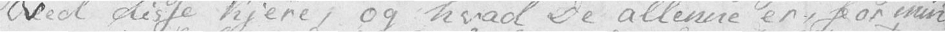ved disse Kjere, og hvad De allenne er, for min
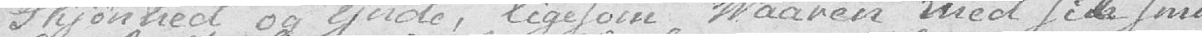Skjønhed og ynde, ligesom Vaaren med sit smi
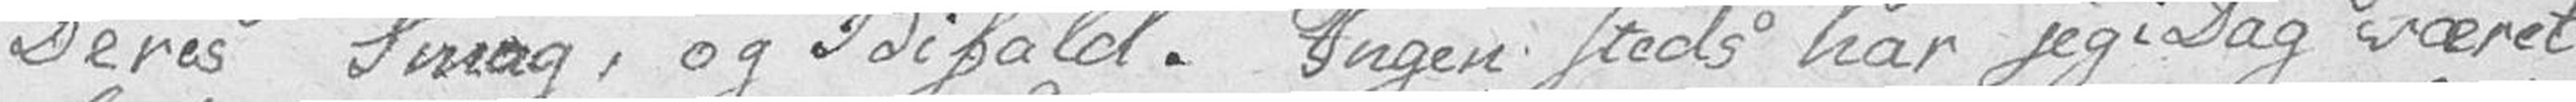Deres Smag, og Bifald. Ingen steds har jeg i Dag været
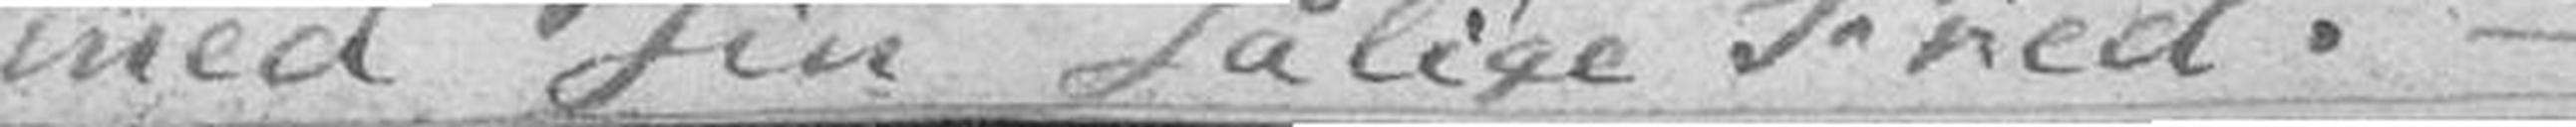med sin salige Fred. –
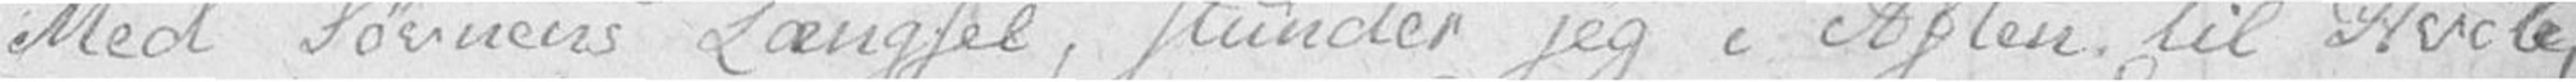Med Søvnens Længsel, stunder jeg i Aften til Hvile,
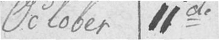October 11de
